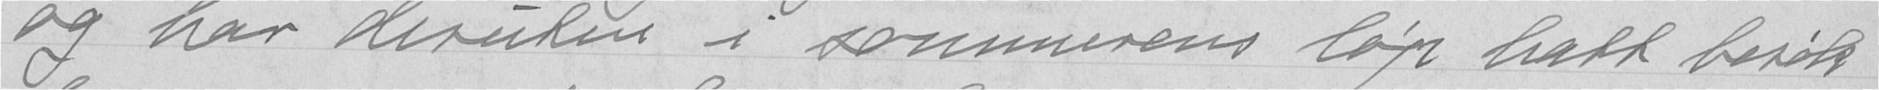og har deruten i sommerens løp hatt besøk
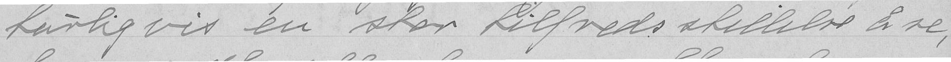turligvis en stor tilfredsstillelse å se,
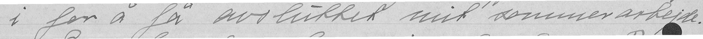i for å få avsluttet mit sommerarbeide.
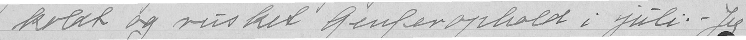koldt og rusket Genserophold i juli.- Jeg
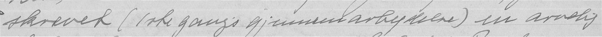skrevet (1ste gangs gjennemarbejdelse) en arvelig-
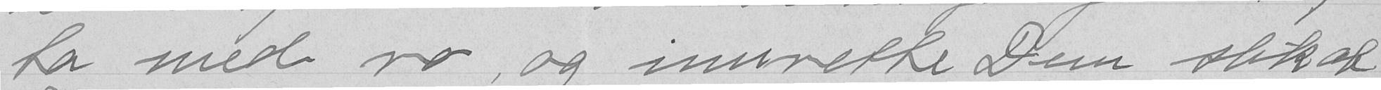ta med ro, og innrette Dem slik at
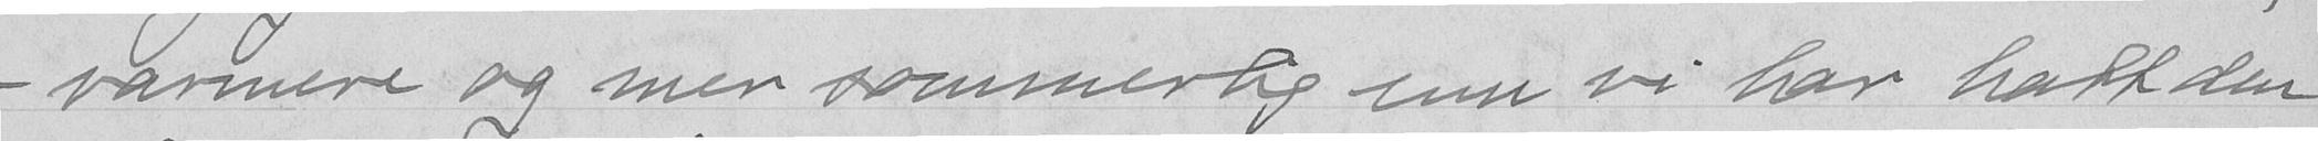- varmere og mer sommerlig enn vi har hatt den
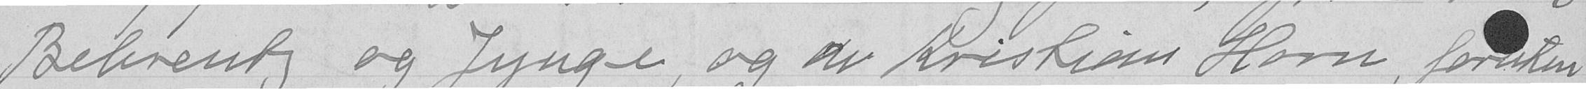Behrentz og Jynge, og av Kristian Horn, foruten
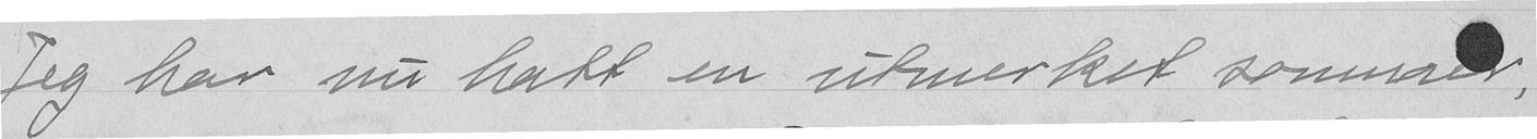Jeg har nu hatt en utmerket sommer.
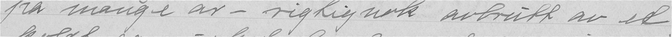på mange år - rigtignok avtrutt av et
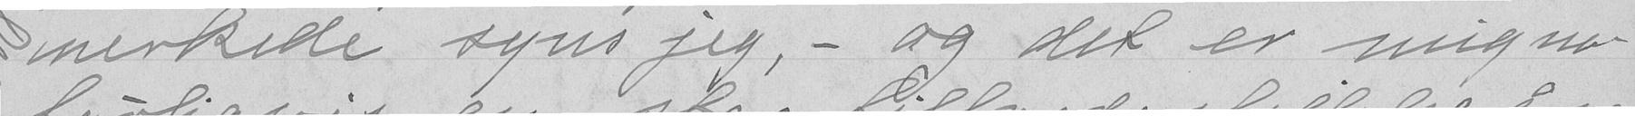merkede syns jeg, - og det er mig na-
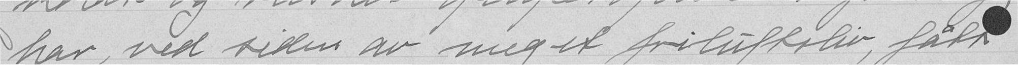har, ved siden av meget friluftsliv, fått
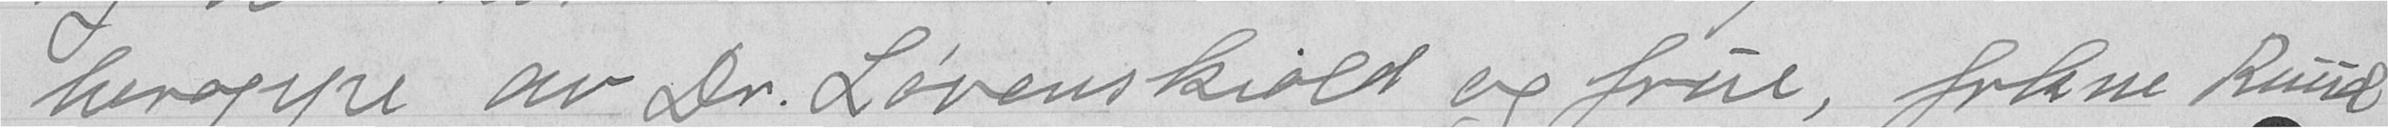heroppe av Dr. Løvenskold og frue, frkne Ruud
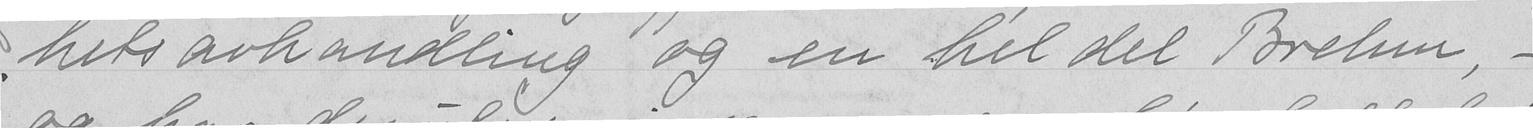hetsavhandling og en hel del Brelun, -
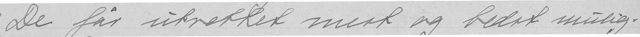De får utrettet mest og bedst mulig.
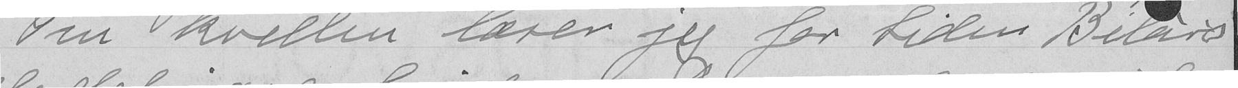Om kvellen læser jeg for tiden Bilars
